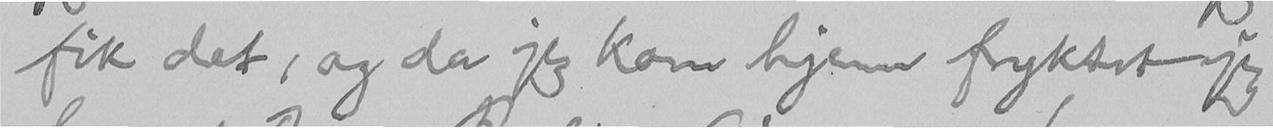fik det, og da jeg kom hjem fryktet jeg
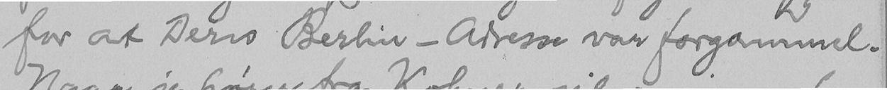for at Deres Berlin-Adresse var forgammel.
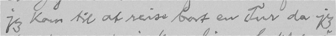jeg kom til at reise bort en Tur da jeg
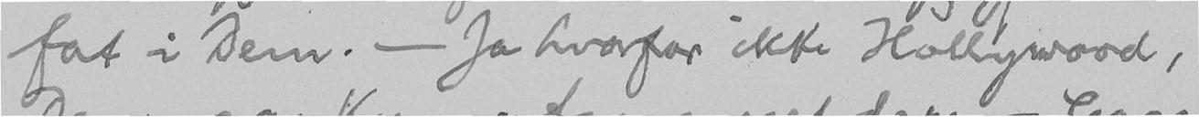fat i Dem. - Ja hvorfor ikke Hollywood,
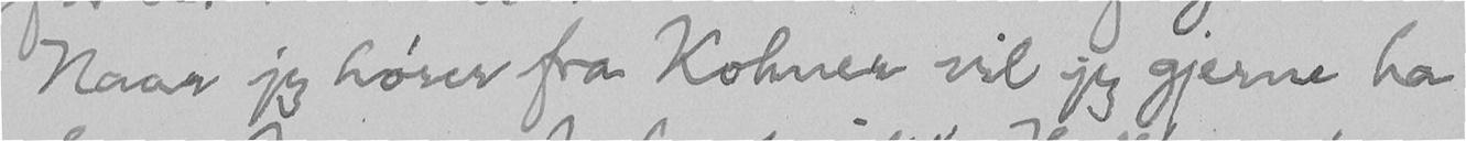Naar jeg hører fra Kohner vil jeg gjerne ha
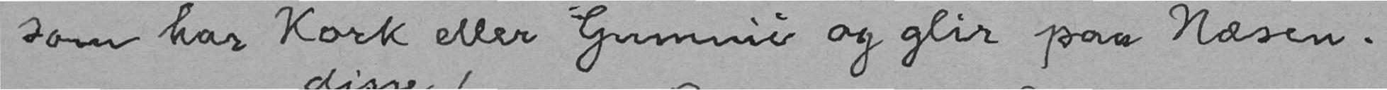som har Kork eller Gummi og glir paa Næsen.
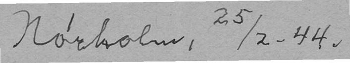Nørholm, 25/2. 44.
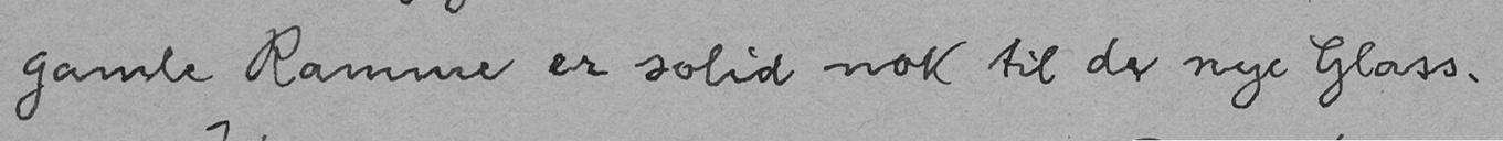gamle Ramme er solid nok til de nye Glass.
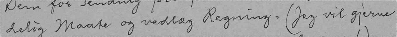delig Maate og vedlæg Regning. (Jeg vil gjerne
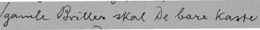gamle Briller skal De bare kaste
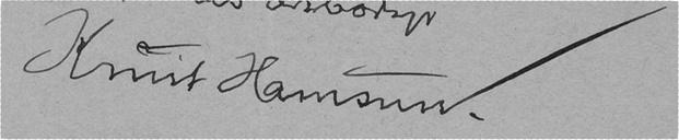Knut Hamsun.
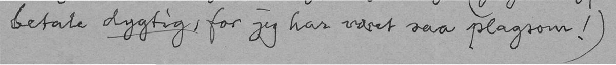betale dygtig, for jeg har været saa plagsom!)
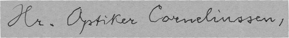Hr. Optiker Corneliussen,
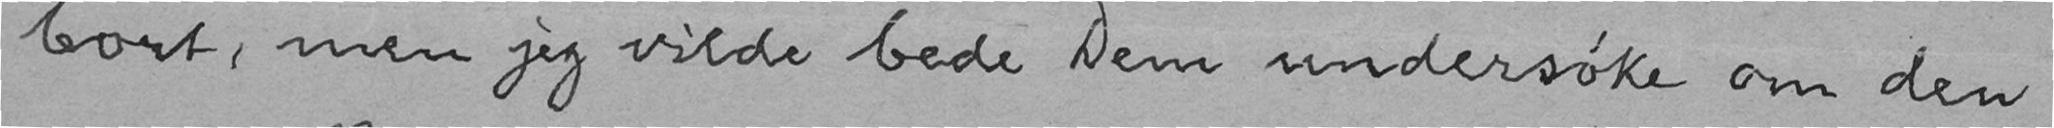bort, men jeg vilde bede Dem undersøke om den
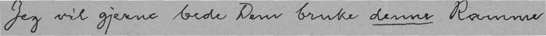Jeg vil gjerne bede Dem bruke denne Ramme
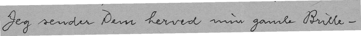Jeg sender Dem herved min gamle Brille-
